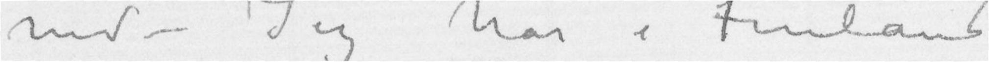ned – Jeg har i Finland
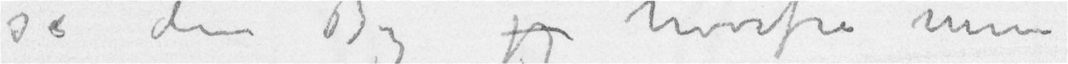se den By jeg hvorfra mine
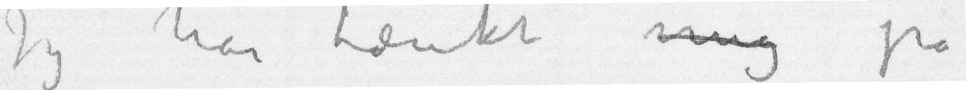Jeg har tænkt mig på
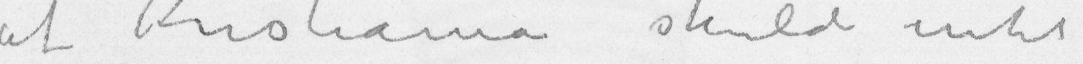af Kristiania skulde intet
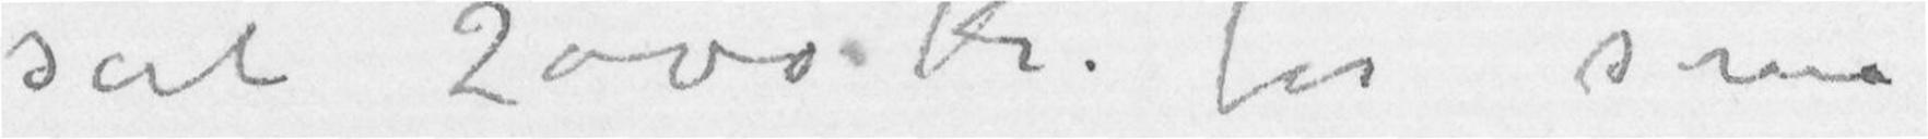sat 2000 Kr. for små
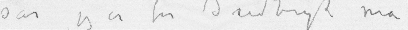sår jeg er for Indtryk må
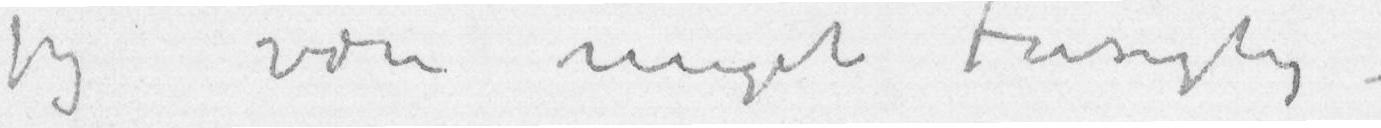jeg være meget forsigtig –
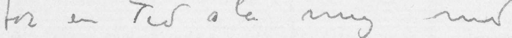for en Tid slå mig ned
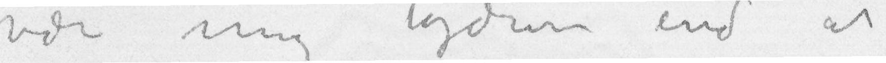være mig kjærere end at
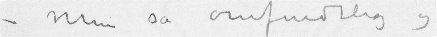– Men så ømfindtlig og
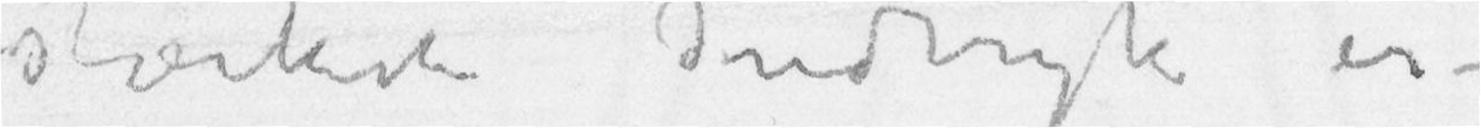stærkeste Indtryk er –
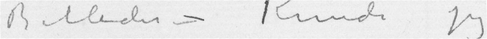Billeder – Kunde jeg
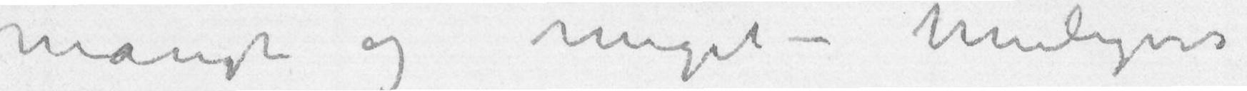mangt og meget – muligvis
